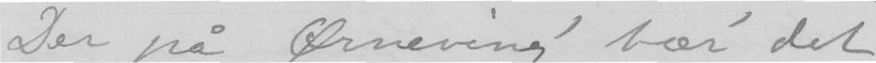Der på Ørneving' bær' det
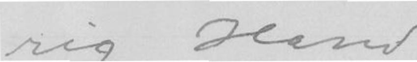rig Hand.
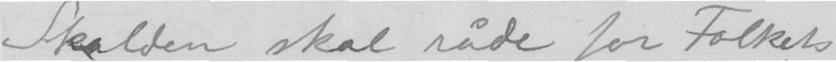Skalden skal råde for Folkets
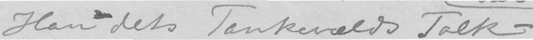Han dets Tankevæld Tolk -
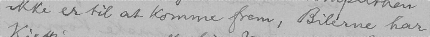ikke er til at komme frem, Bilerne har
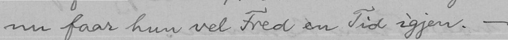nu faar hun vel Fred en Tid igjen. -
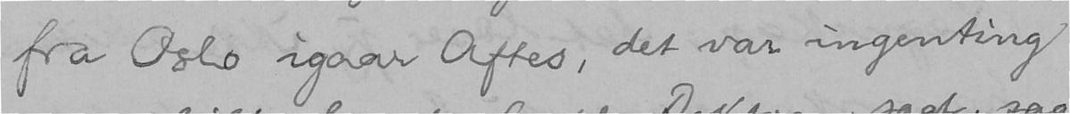fra Oslo igaar Aftes, det var ingenting
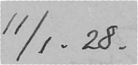11/1. 28.
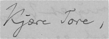Kjære Tore,
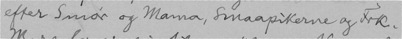efter Smør og Mama, Smaapikerne og Frk.
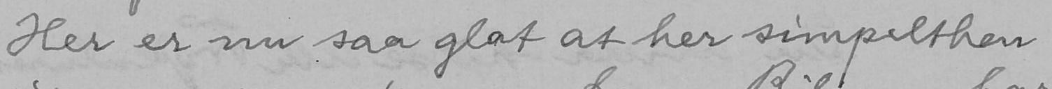Her er nu saa glat at her simpelthen
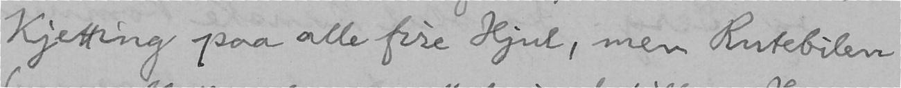Kjetting paa alle fire Hjul, men Rutebilen
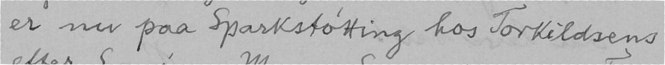er nu paa Sparkstøtting hos Torkildsens
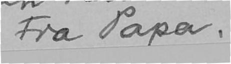Fra Papa.
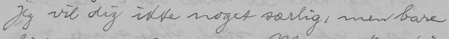jeg vil dig ikke noget særlig, men bare
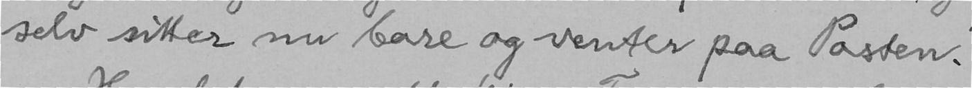selv sitter nu bare og venter paa Posten.
